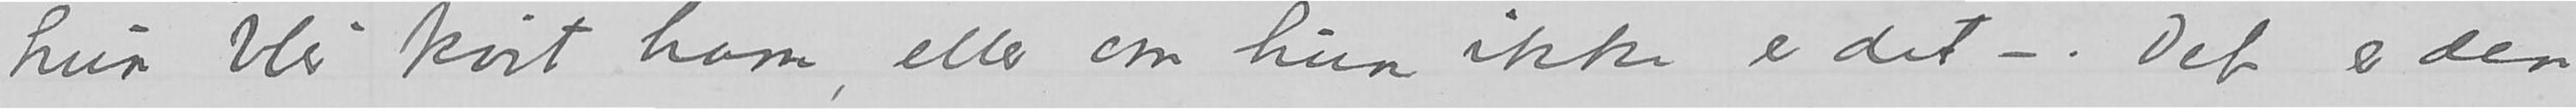hun blir kvit ham, eller om hun ikke er det –. Det er den
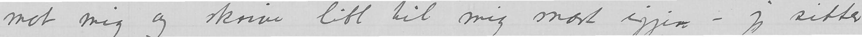mot mig og skrive litt til mig snart igjen – jeg sitter
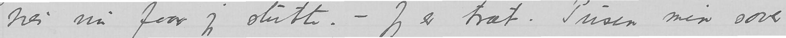Nei nu faar jeg slutte. – Jeg er træt. Pusen min sover
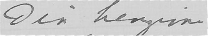Din hengivne
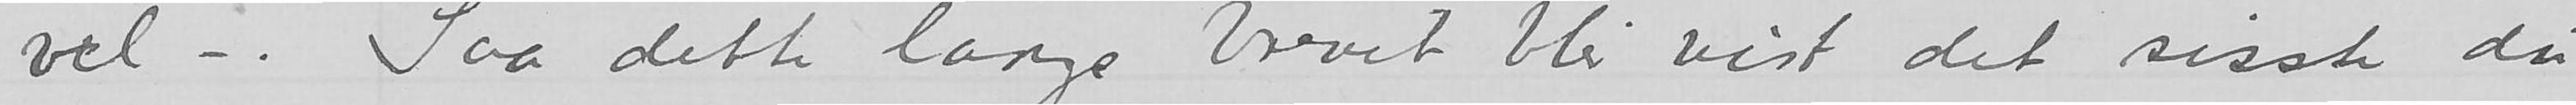vel –. Saa dette lange brevet blir vist det sisste du
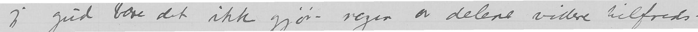jeg gud bare det ikke gjør nogen av delene videre tilfreds-
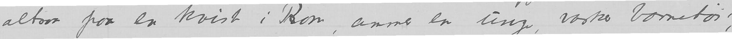altsaa paa en kvist i Rom, ammer en unge, vasker barnetøi,
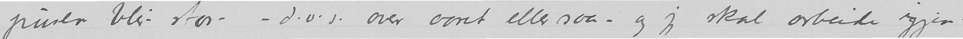pusen blir stor – d.v.s. over aaret eller saa – og jeg skal arbeide igjen.
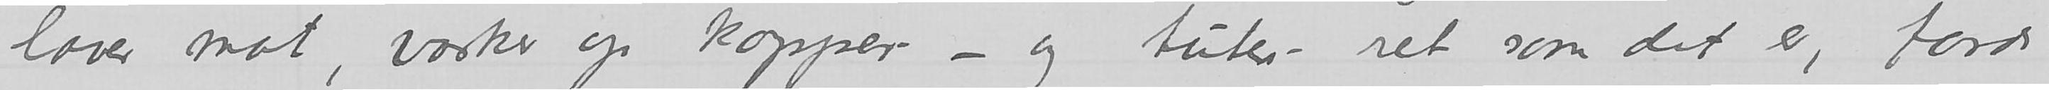laver mat, vasker op kopper – og tuter ret som det, fordi
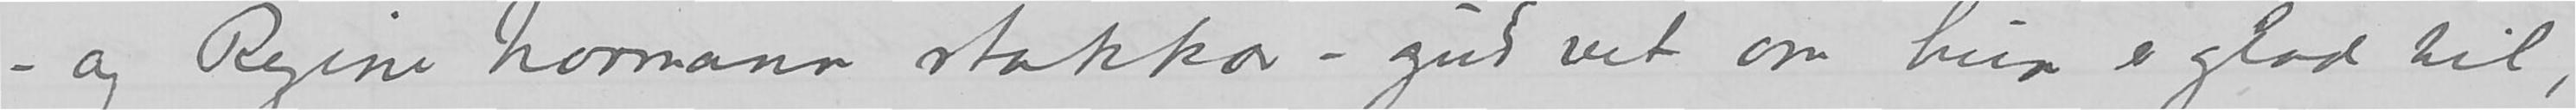–og Regine Normann stakkar – gud vet om hun er glad til,
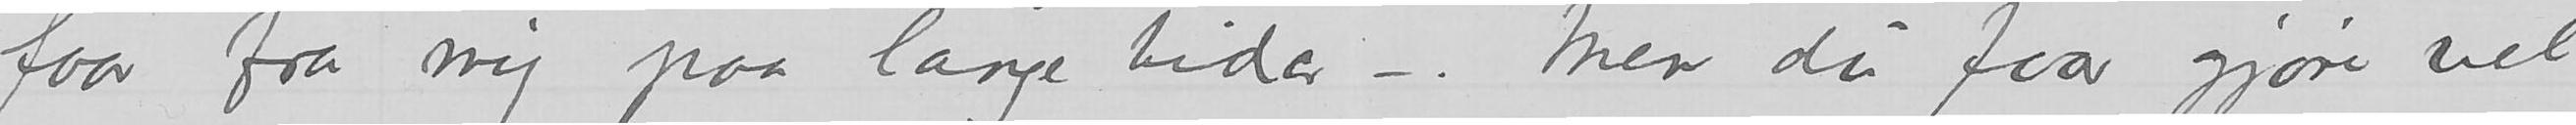faar fra mig paa lange tider –. Men du faar gjøre vel
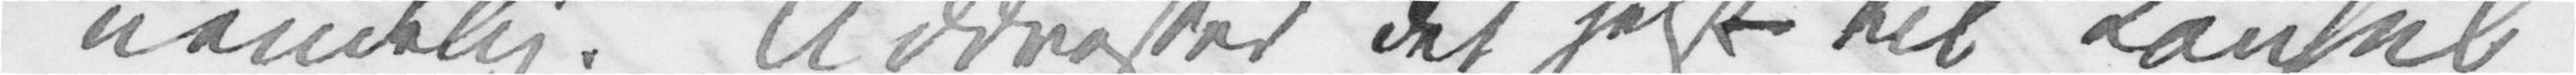uendelig. Addresser det helst til Konsul
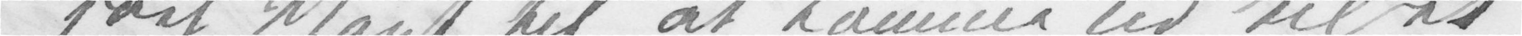fået Magt til at komme ud til et
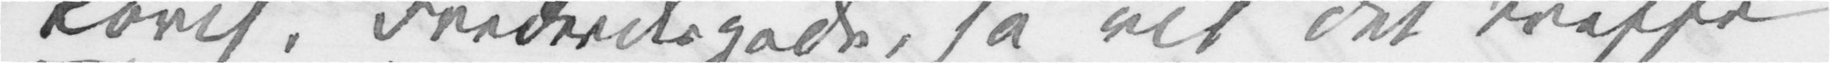Lorch, Fredricksgade, så vil det treffe
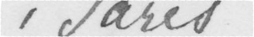i Paris
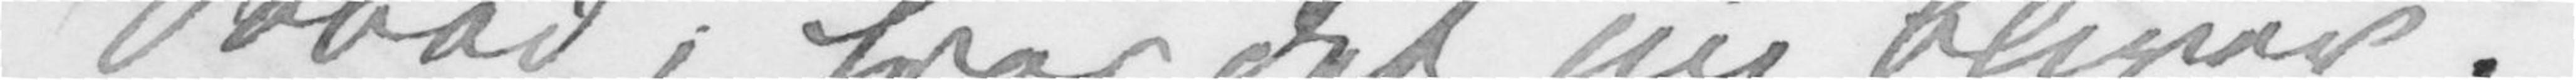Søbad, hvor det nu bliver.
